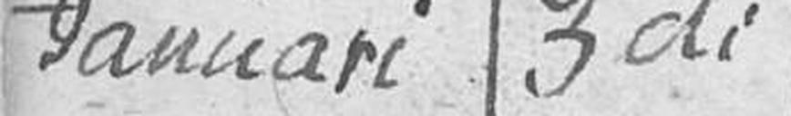Januari 3di
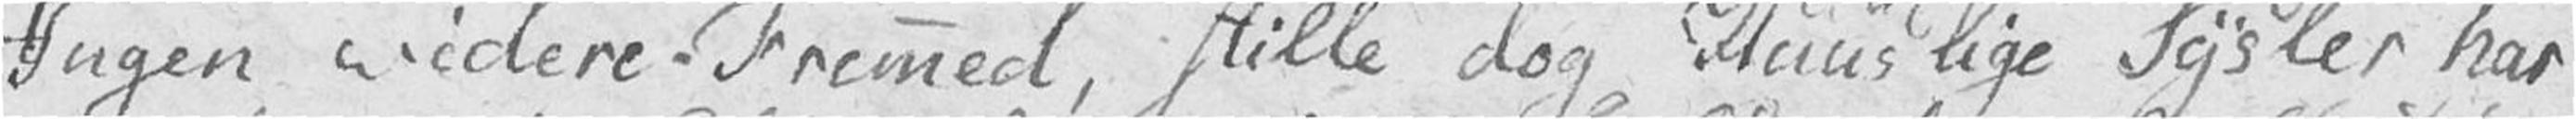Ingen videre Fremmed, stille dog Huuslige Sysler har
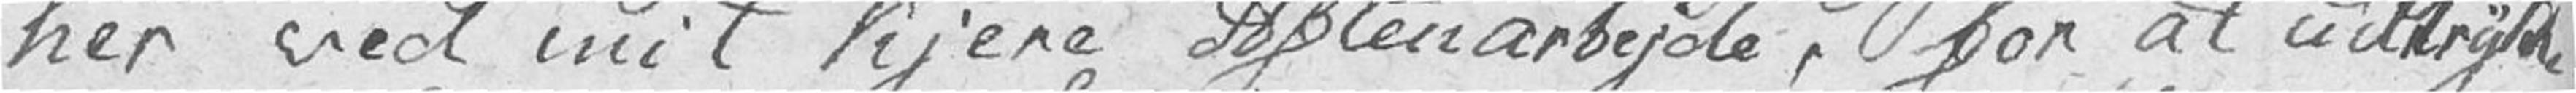her ved mit kjere Aftenarbejde, for at udtrykke
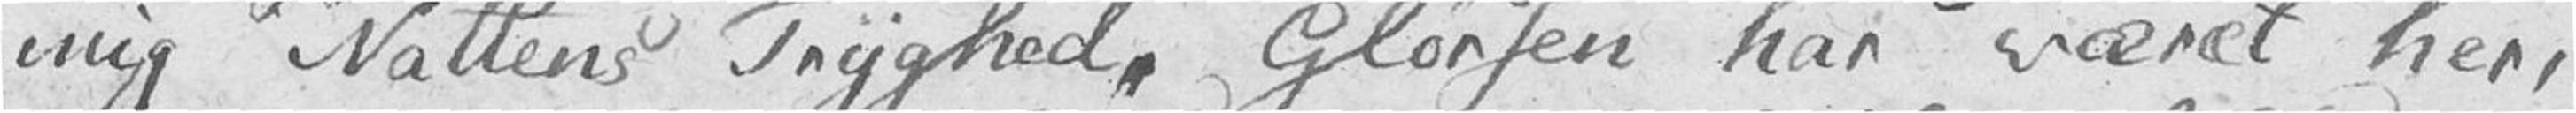mig Nattens Tryghed. Glørsen har været her,
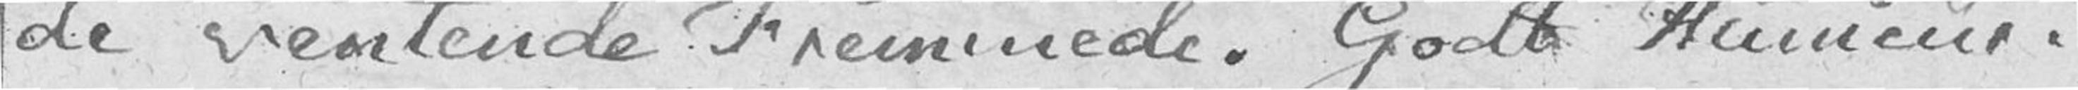de ventende Fremmede. Godt Humeur.
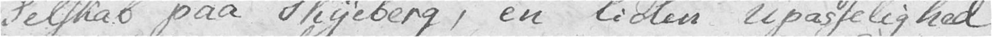Selskab paa Skyeberg, en liden upasselighed
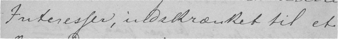Interesser, indskrænket til et
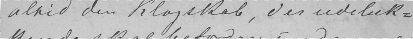altid den Klogskab, der udeluk-
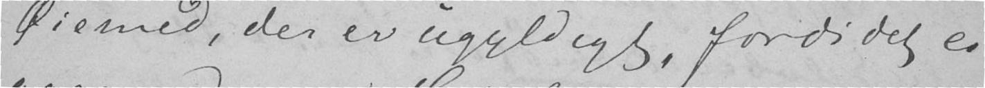Øiemed, der er ugyldigt fordi det ei
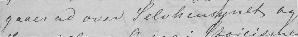gaaer ud over Selvhensynet og
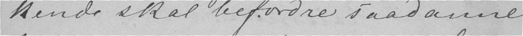kende skal befordre saadanne
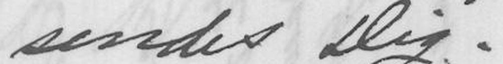sendes Dig.
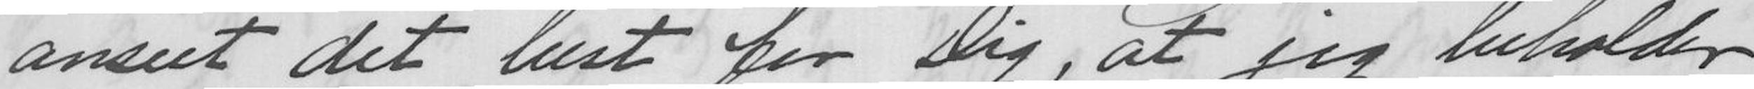anseet det best for Dig, at jeg beholder
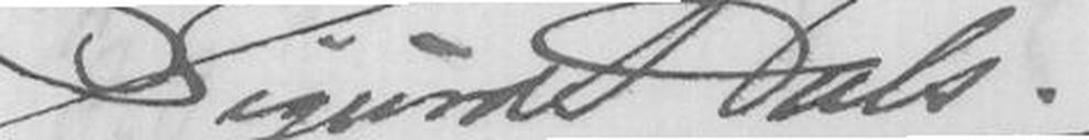Sigurd Hals.
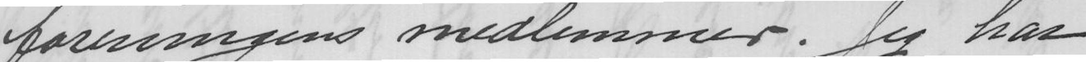forreningens medlemmer. Jeg har
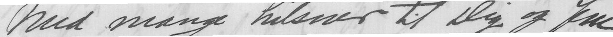Med mange hilsener til Dig og fru
